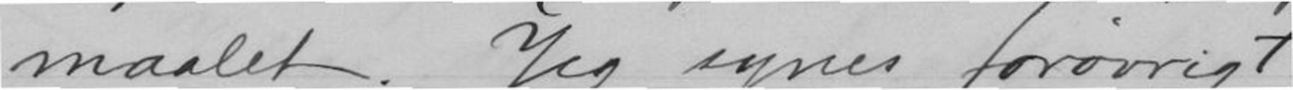maalet. Jeg synes forøvrig
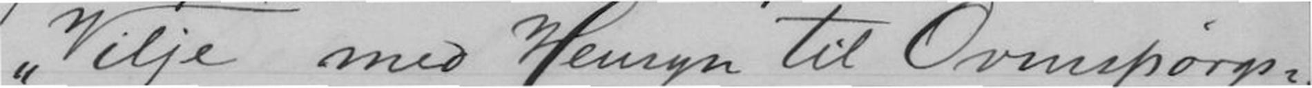"Vilje" med Hensyn til Ovnespørgs-
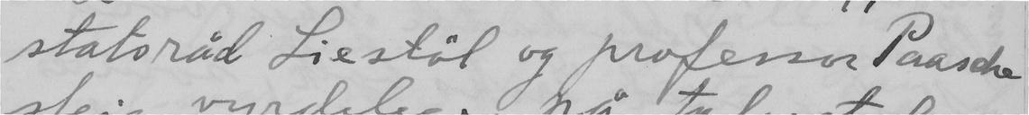statsråd Liestöl og professor Paasche
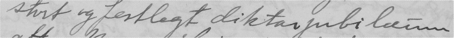stort og festlegt diktarpublikum
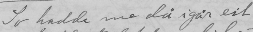So hadde me då igår eit
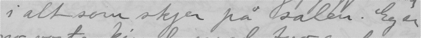i alt som skjer på salen. Eg er
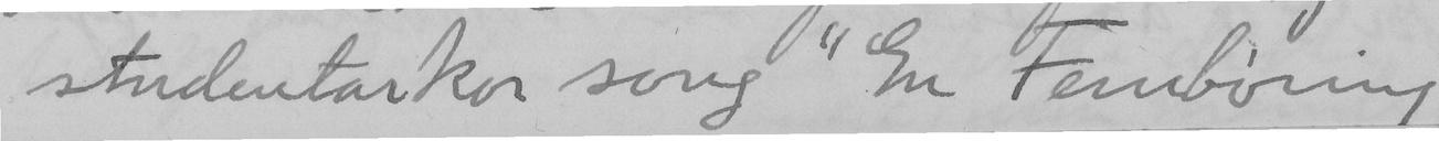studentarkor song "Ein Femböring"
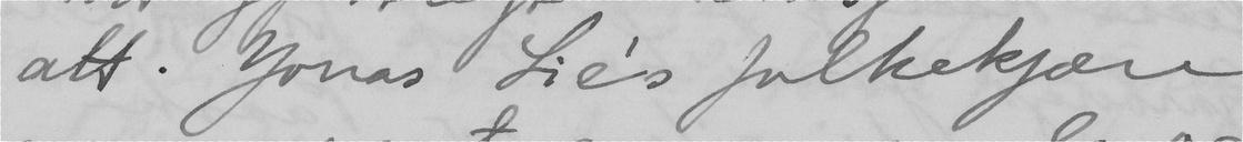att. Jonas Lie's folkekjære
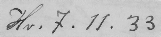Hv. 7.11.33
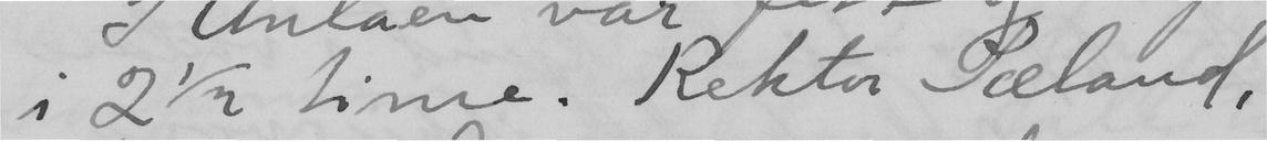i 2 1/2 time. Rektor Sæland,
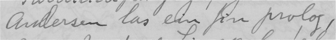Andersen las en fin prolog,
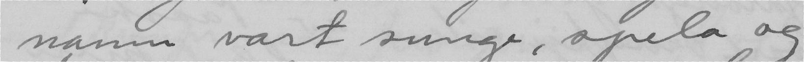namn vart sunge, spela og
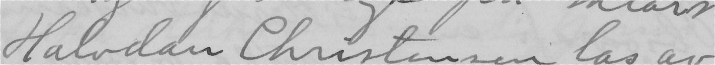Halvdan Christensen las av
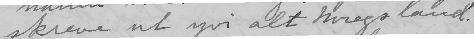skreve ut yvi alt Noregs land.
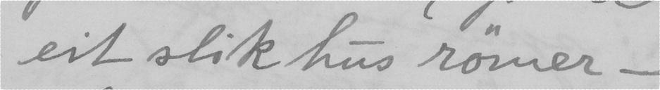eit slik hus römer –
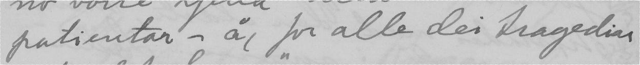patientar – å, for alle dei tragediar
